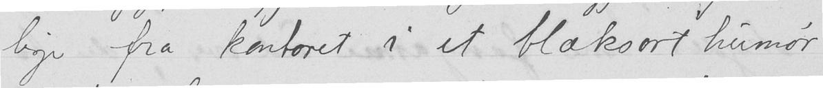lige fra kontoret i et blæksort humør
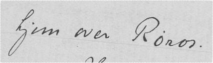hjem over Røros.
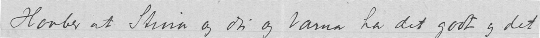Haaber at Stina og du og barna har det godt og det
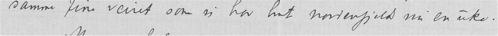samme fine veiret som vi har hat nordenfjeld nu en uke.
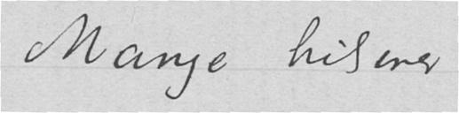Mange hilsener
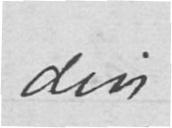din
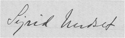Sigrid Undset
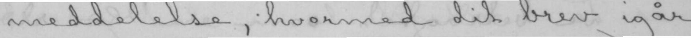meddelelse, hvormed dit brev igår
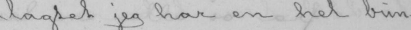Uagtet jeg har en hel bun-
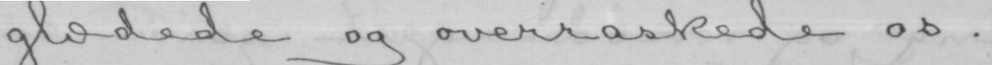glædede og overraskede os.
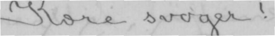Kære svoger!
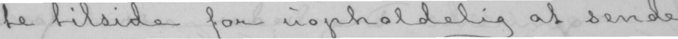te tilside for uopholdelig at sende
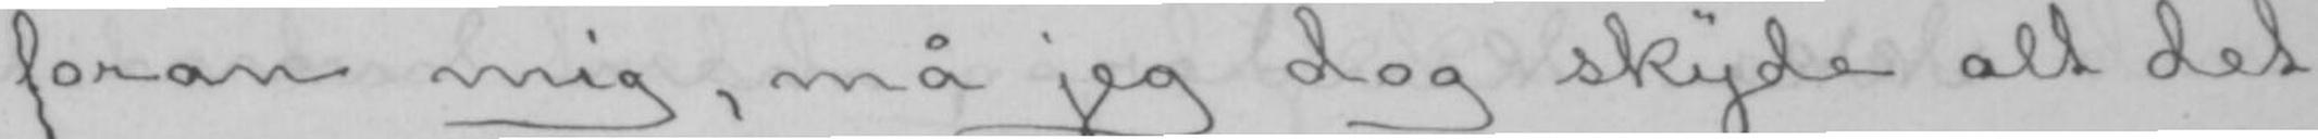foran mig, må jeg dog skyde alt det-
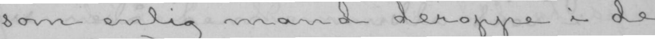som enlig mand deroppe i de
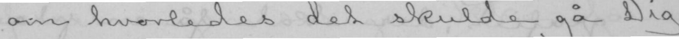om hvorledes det skulde gå Dig
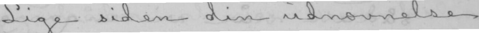Lige siden din udnævnelse
